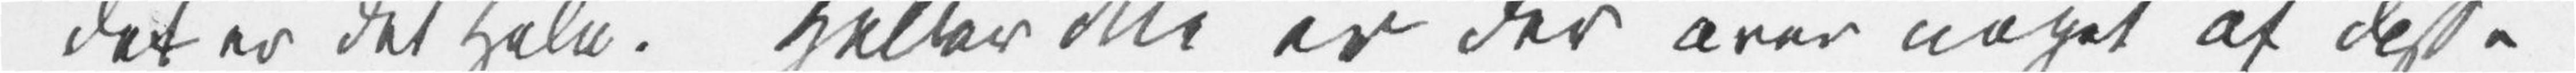det er det Hele. Heller ikke er der over noget af disse
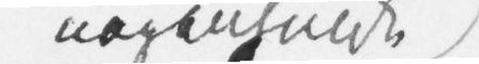nogensinde
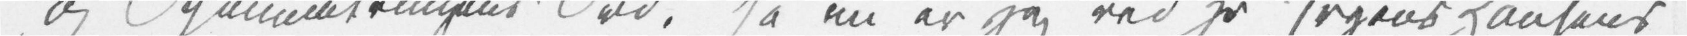og Opmuntringens Ord, så nu er jeg ved Hr Irgens Hansens
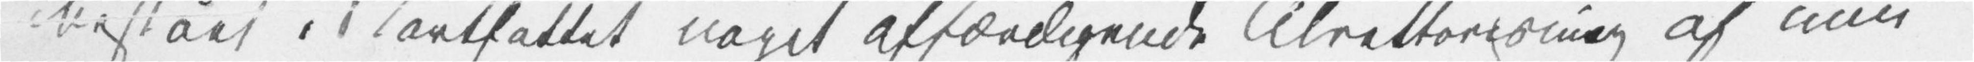bestået i kortfattet noget affærdigende Tilrettevisning af min
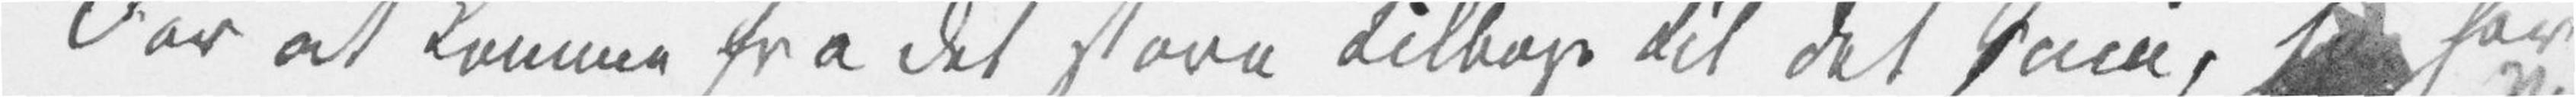For at komme fra det store tilbage til det små, så har
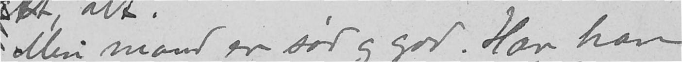Min mand er sød og god. Har han
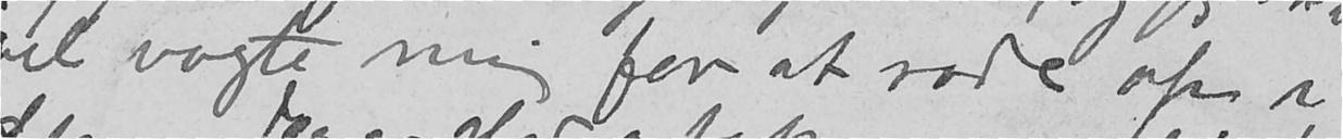vel vogte mig for at rode op i
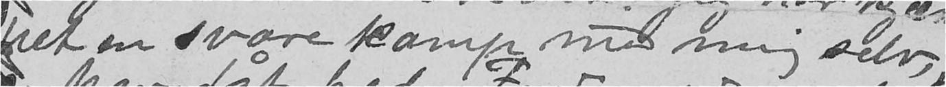pet en svare kamp med mig selv,
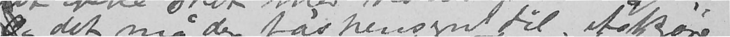Og det må der ta's hensyn til. Å kjære
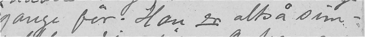gange før. Han er altså sim-
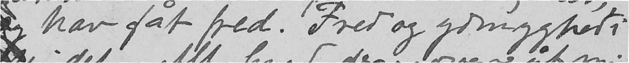og har fåt fred. Fred og ydmyghed i
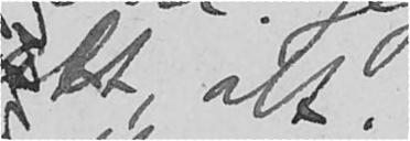alt, alt.
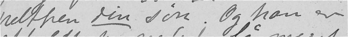pelthen din søn. Og han er
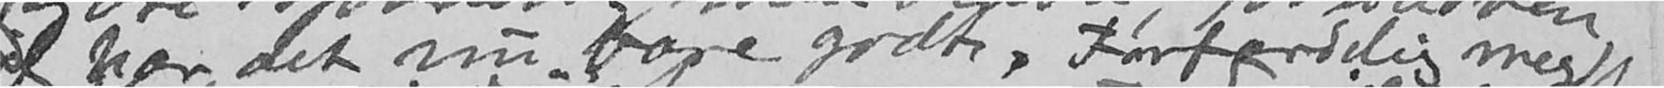Jeg har det nu bare godt. Forfærdelig meget
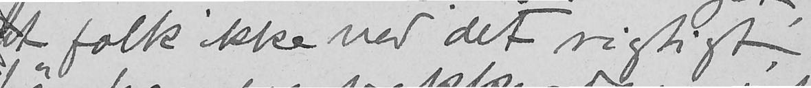at folk ikke ved det rigtigt,
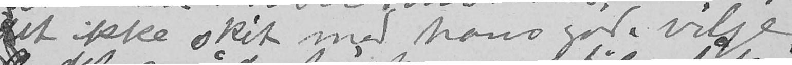det ikke skét med hans gode vilje.
